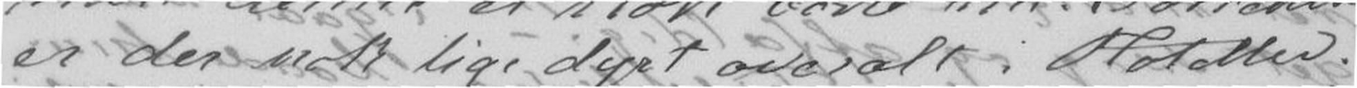er der nok lige dyrt overalt i Hoteller.
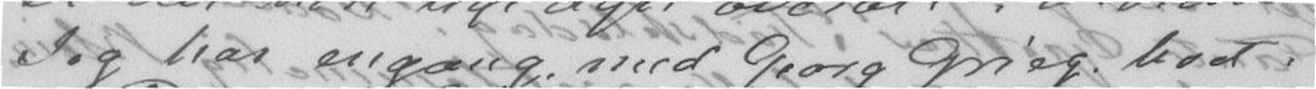Jeg har engang med Goerg Grieg boet i
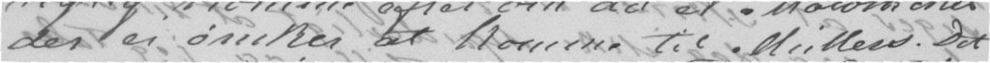der ei ønsker at komme til Müllers. Det
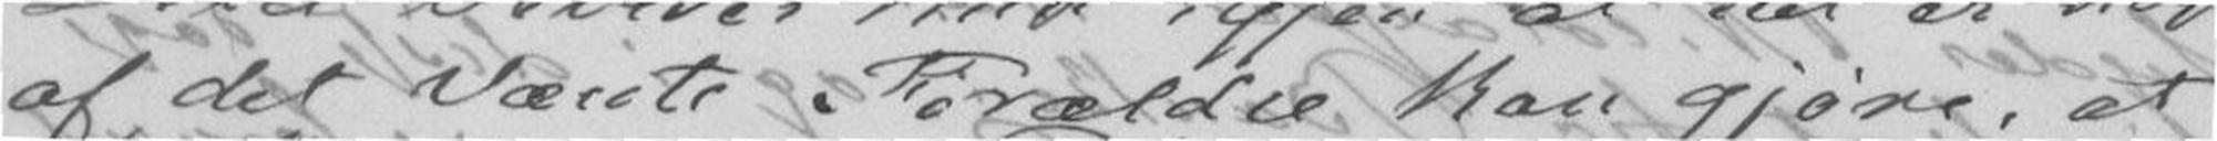af det værste Forældre kan gjøre, at
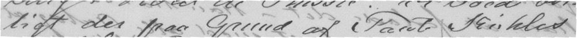ligt der paa Grund af Tante Kühles
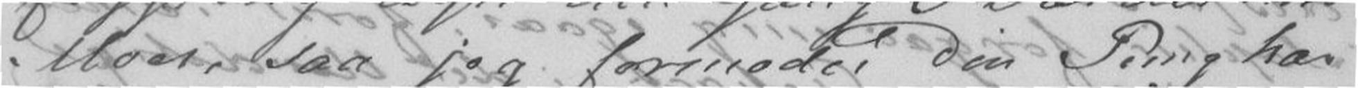Moer, saa jeg formoder Din Pung har
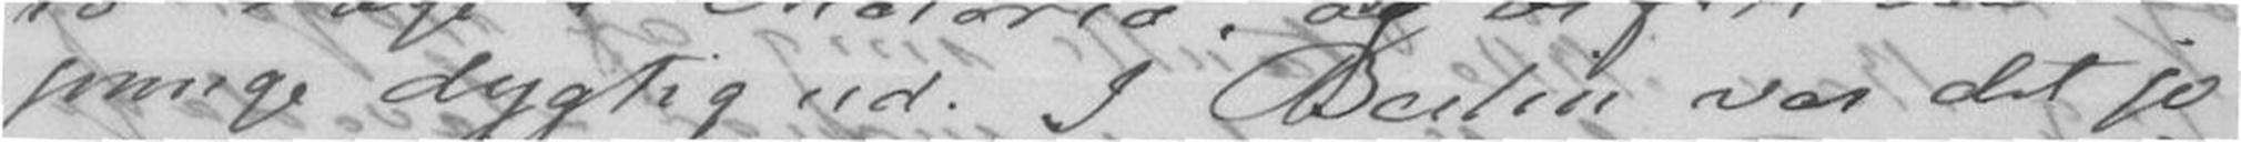punge dygtig ud. I Berlin var det jo
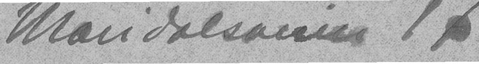Maridalsveien 17
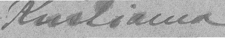Kristiania
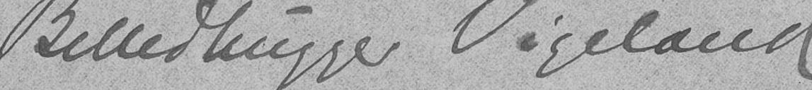Billedhugger Vigeland
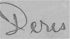Deres
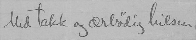Med takk og ærbødig hilsen
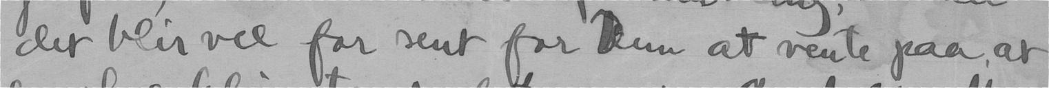det blir vel for sent for Dem at vente paa, at
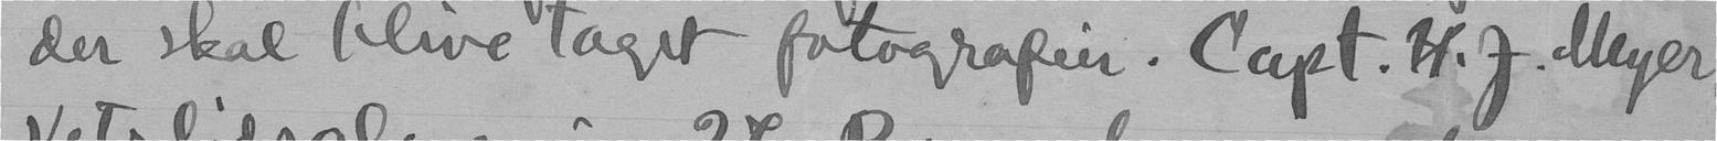der skal blive taget fotografier.. Capt. H. J. Meyer
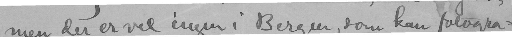men der er vel ingen i Bergen, som kan fotogra-
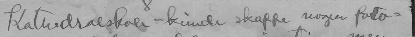Kathedralskole - kunde skaffe nogen foto-
